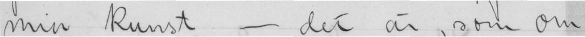min kunst - det är, som om
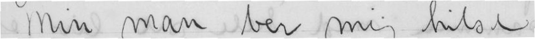Min man ber mig hilse
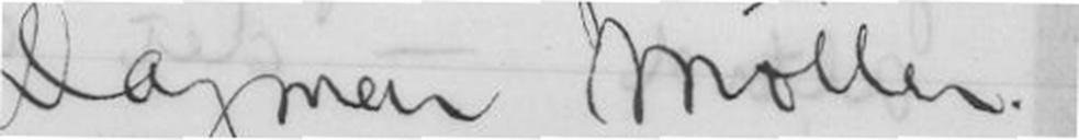Dagmar Møller.
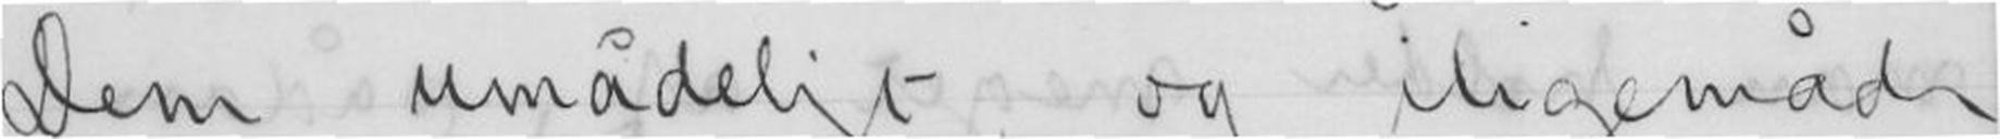Dem umådeligt, og iligemåde
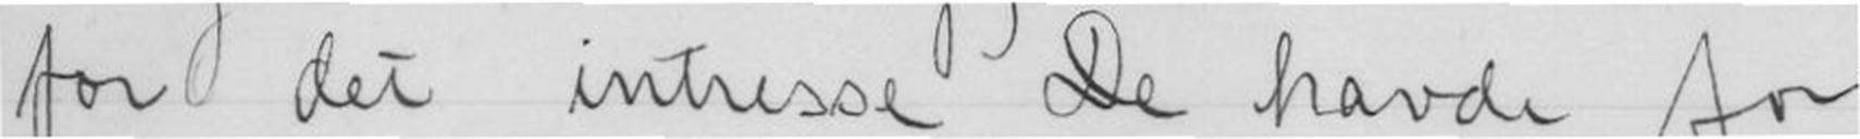for det intresse De havde for
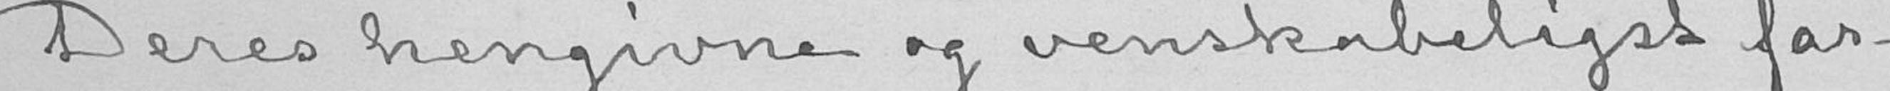Deres hengivne og venskabeligst for-
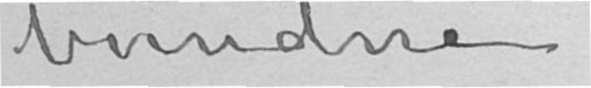bundne
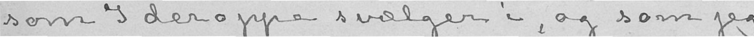som I deroppe svælger i, og som jeg
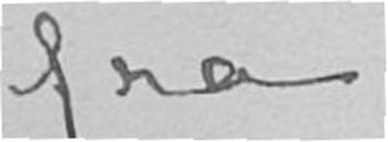fra
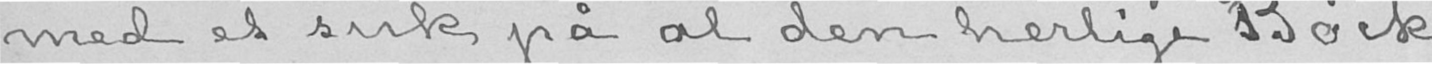med et suk på al den herlige Bock,
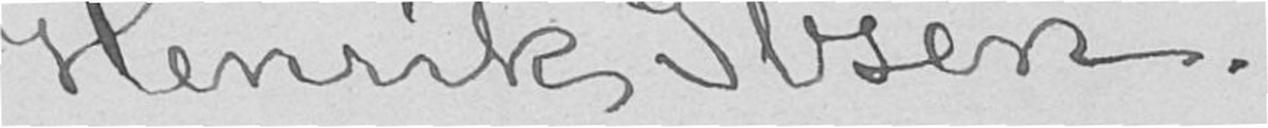Henrik Ibsen.
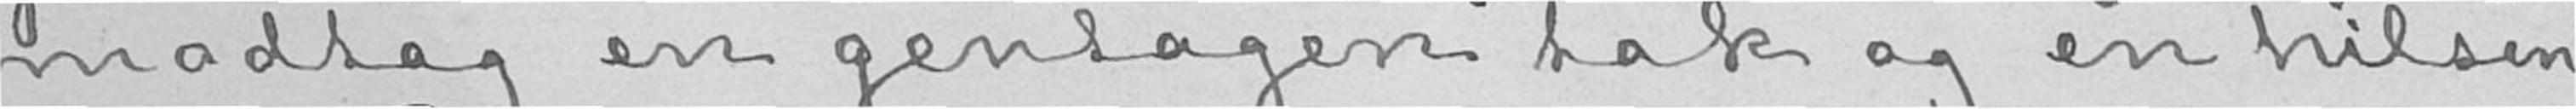modtag en gentagen tak og en hilsen
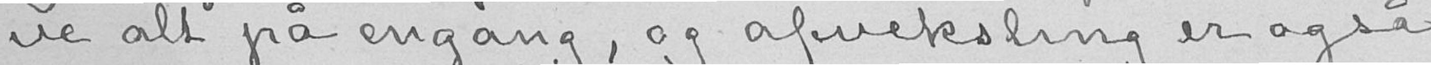ve alt på engang, og afveksling er også
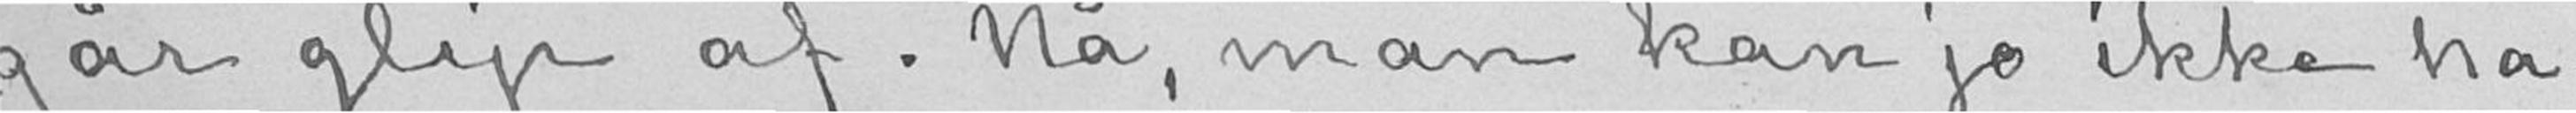går glip af. Nå, man kan jo ikke ha-
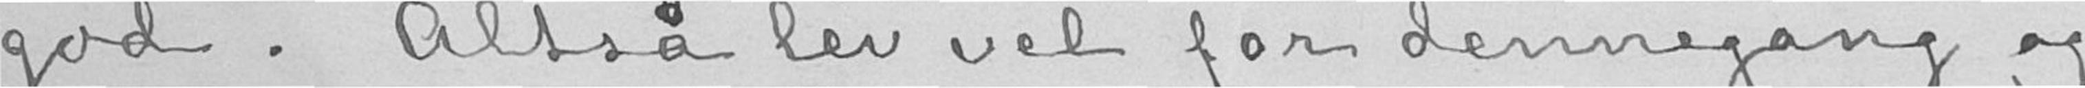god. Altså lev vel for dennegang og
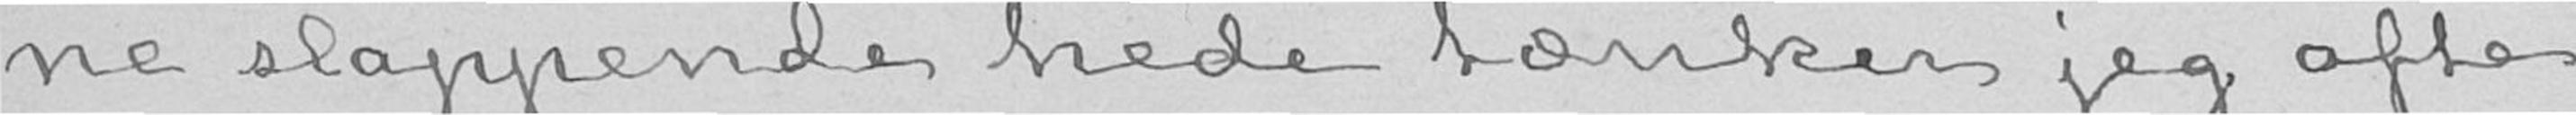ne slappende hede tænker jeg ofte
